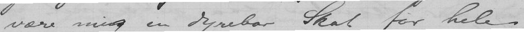være mig en dyrebar Skat for hele
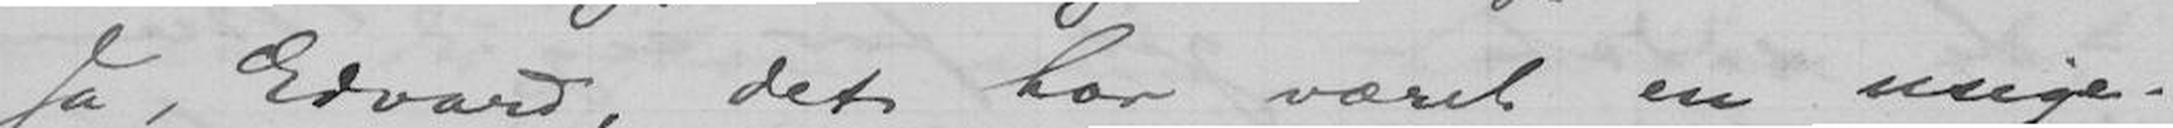Ja, Edvard, det har været en usige-
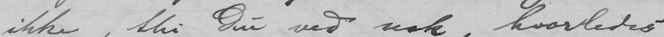ikke, thi Du ved nok, hvorledes
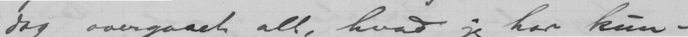dog overgaaet alt, hvad jeg har kun-
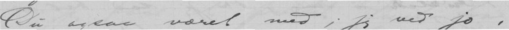Du ogsaa været med; jeg ved jo,
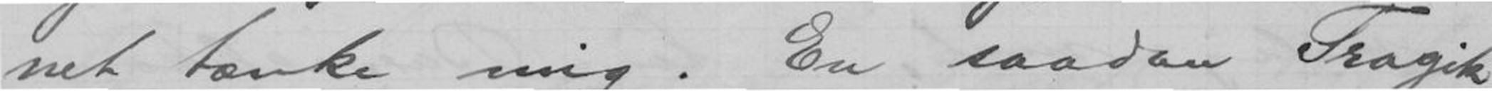net tænke mig. En saadan Tragik,
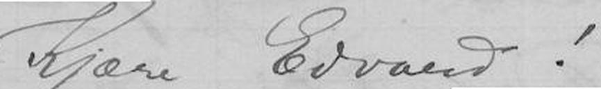Kjære Edvard!
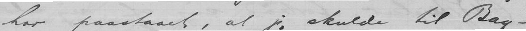har paastaaet, at jeg skulde til Bay-
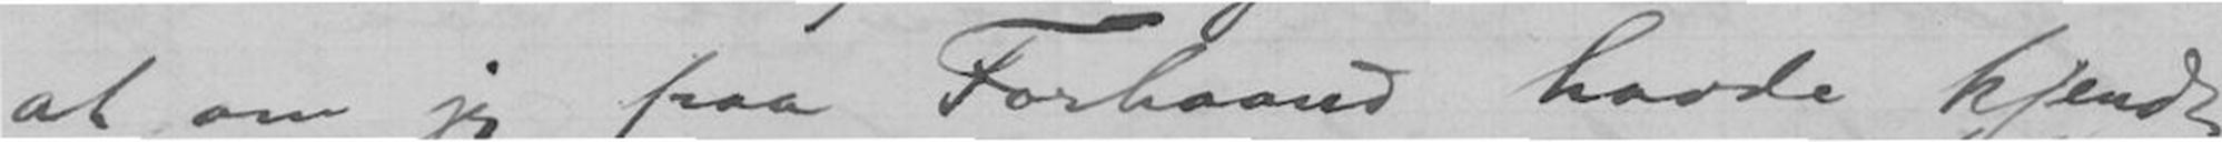at om jeg paa Forhaand havde kjendt
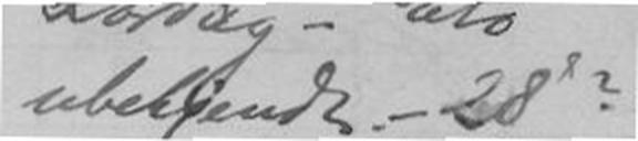ubekjendt - 28 ?
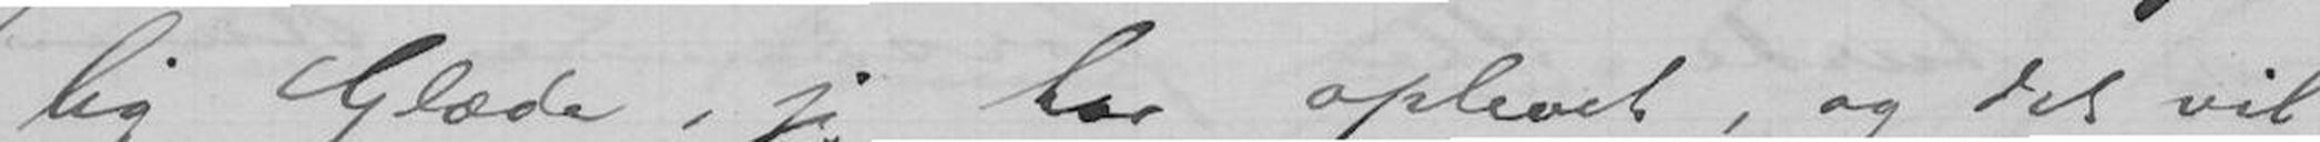lig Glæde, jeg har oplevet, og det vil
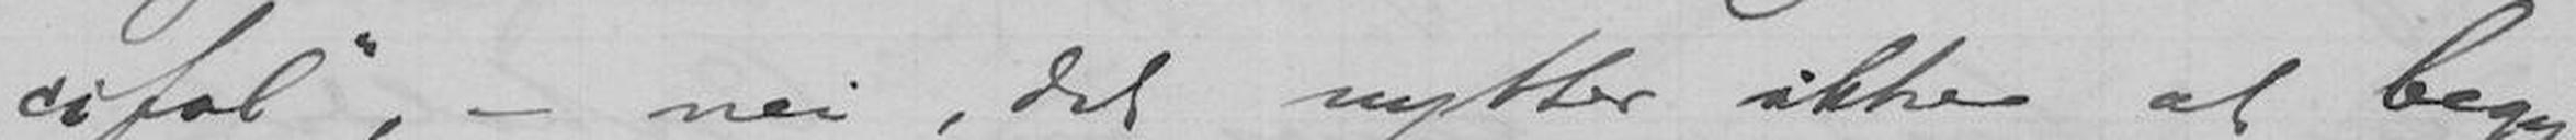cifal", - nei, det nytter ikke at begyn-
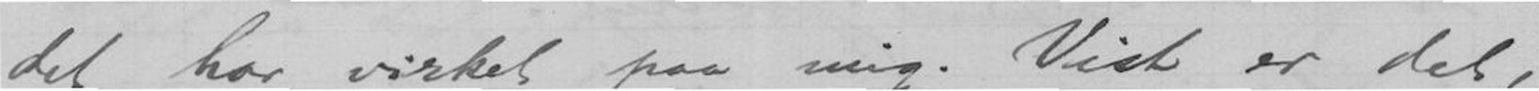det har virket paa mig. Vist er det,
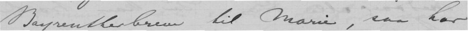Bayreutherbreve til Marie, saa har
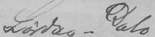Lørdag - Dato
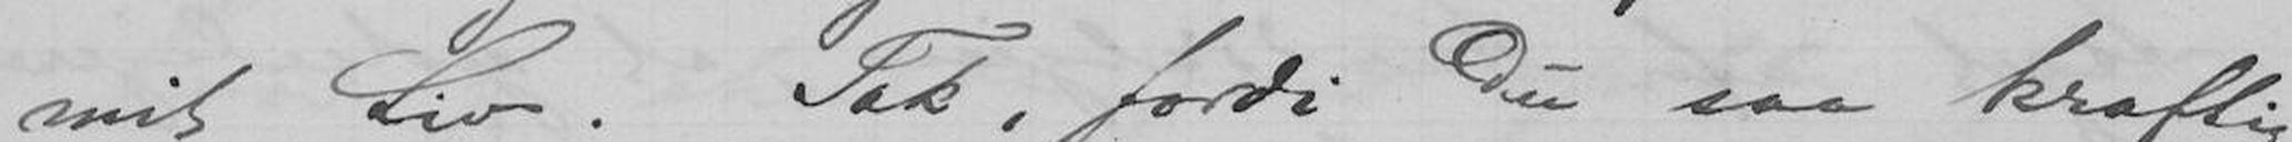mit Liv. Tak, fordi Du saa kraftig
